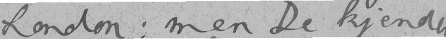London; men De kjender
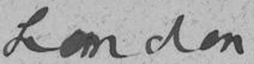London
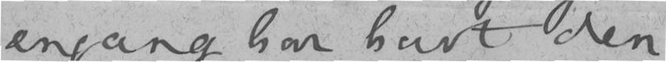engang har havt den
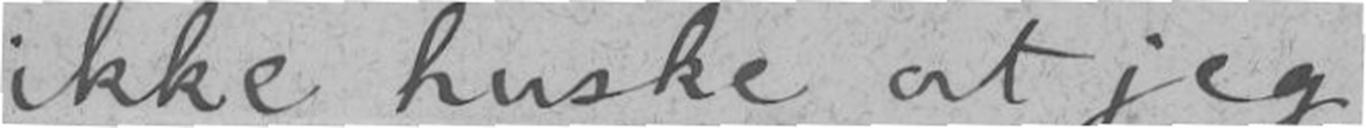ikke huske at jeg
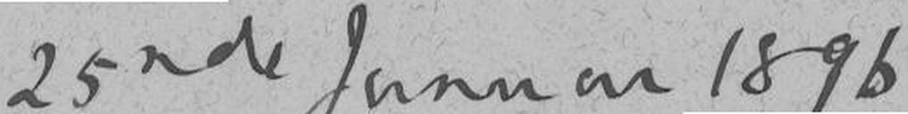25nde Januar 1896
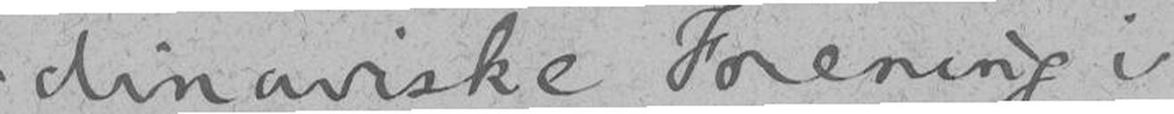dinaviske Forening i
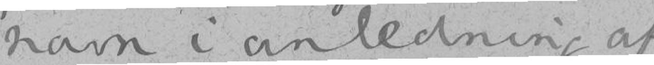navn i anledning af
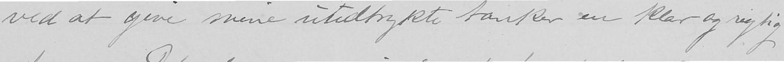ved at give mine uudtrykte tanker en klar og rigtig
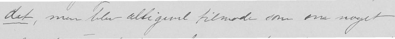det, men blev alligevel tilmode som om noget
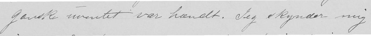ganske uventet var hændt. Jeg skynder mig
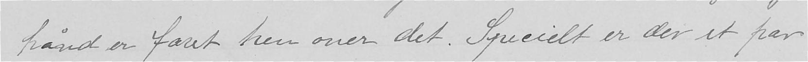hånd er faret hen over det. Specielt er der et par
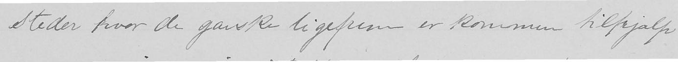steder hvor de ganske ligefrem er kommen tilhjælp
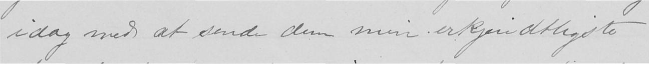idag med at sende dem min erkjendtligste
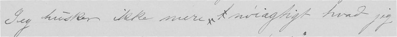Jeg husker ikke mere l nøiagtigt, hvad jeg
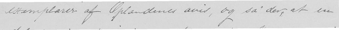exemplarer af Oplandenes avis, og så der, at en
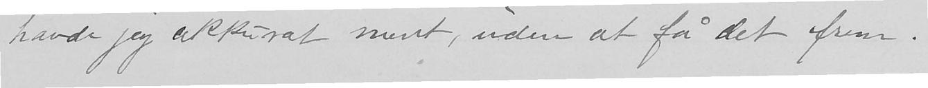havde jeg akkurat ment, uden at få det frem.
